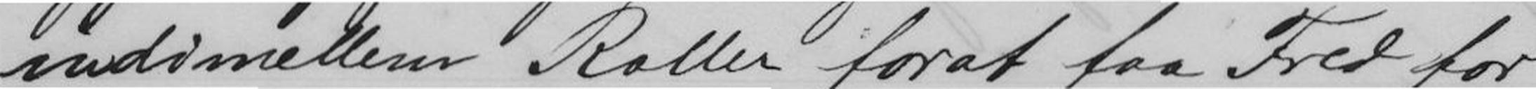indimellem Roller forat faa Fred for
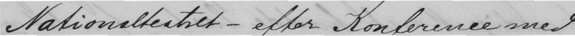Nationalteatret - efter Konference med
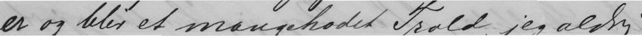er og blir et mangehodet Trold, jeg aldrig
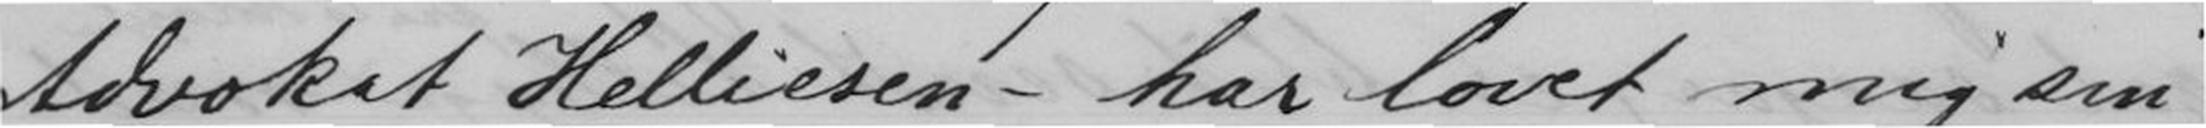Advokat Helliesen - har lovet mig sin
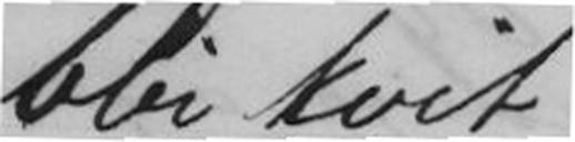blir kvit.
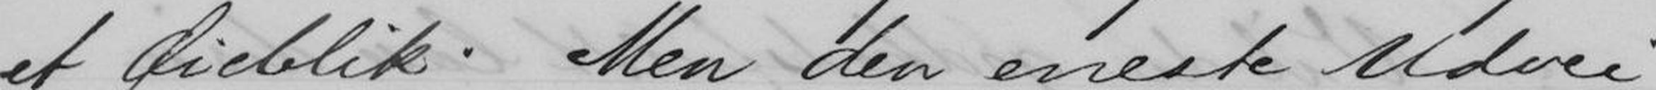et Øieblik. Men den eneste Udvei
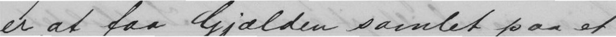er at faa Gjælden samlet paa et
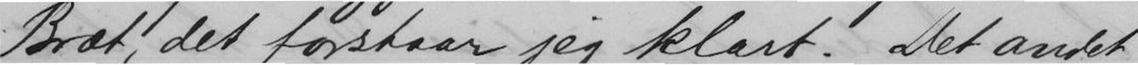Bræt, det forstaar jeg klart. Det andet
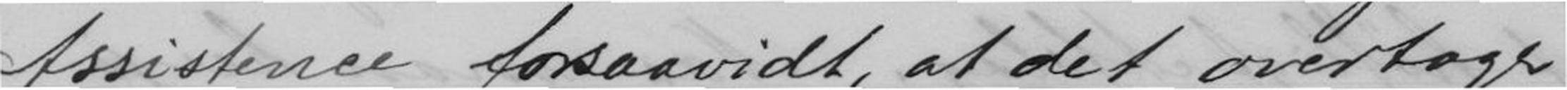Assistence forsaavidt, at det overtager
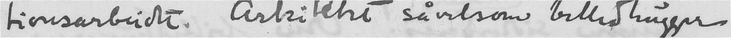tionsarbeidet. Arkitekt såvelsom billedhugger
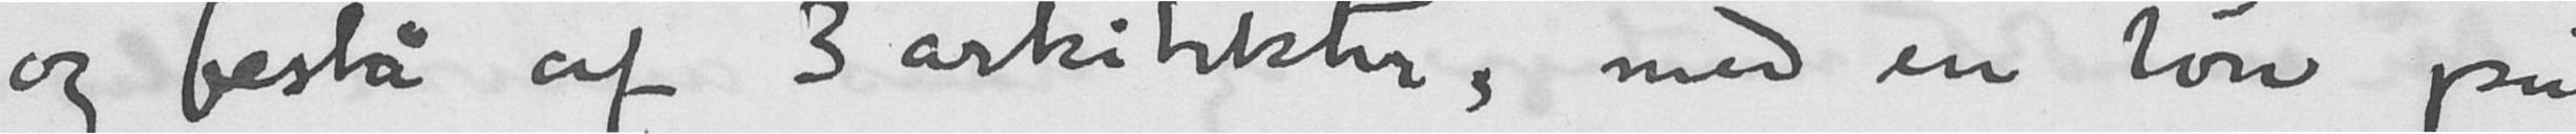og bestå af 3 arkitekter, med en løn på
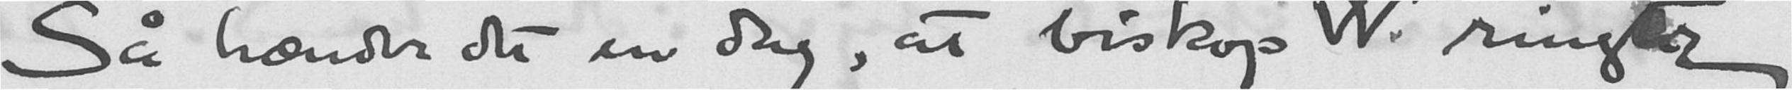Så hænder det en dag, at biskop W. ringte
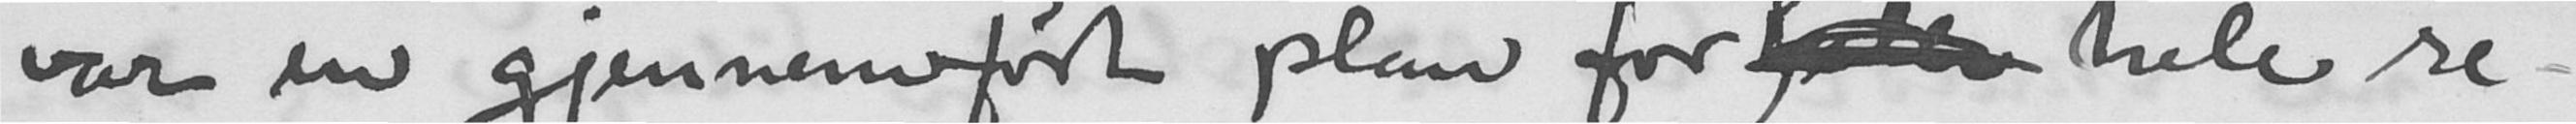var en gjennomført plan for hele hele re-
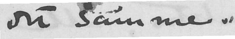det samme.
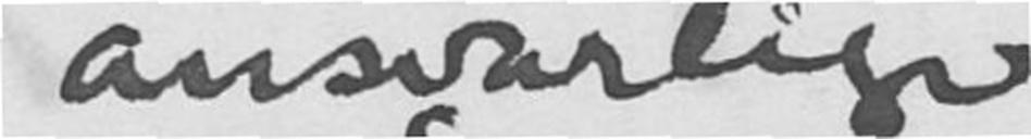ansvarlige
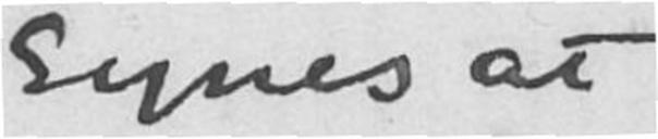synes at
